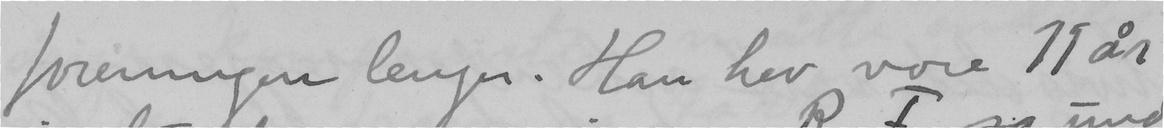foreningen lenger. Han hev vore 11 år
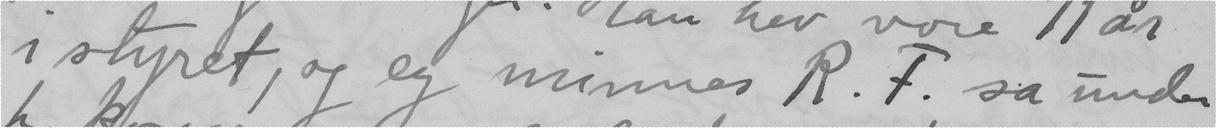i styret, og eg minnes R.F. sa under
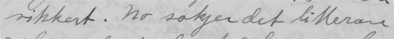sikkert. No sökjer det litterære
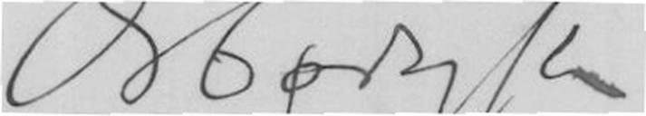Ærbødigst
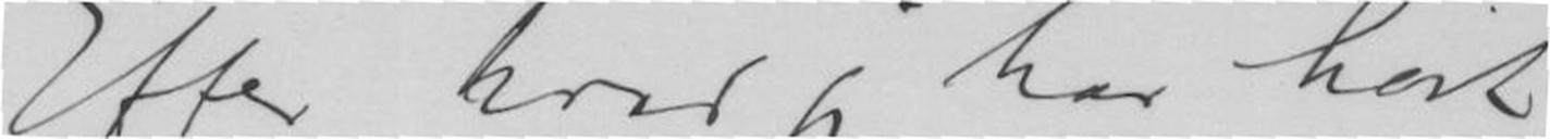Efter hvad jeg har hørt
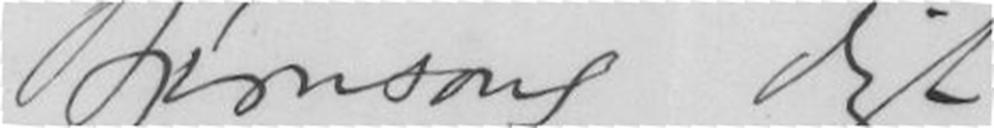Bjørnsons digt.
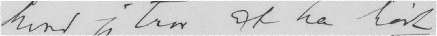hvad jeg tror at ha hørt
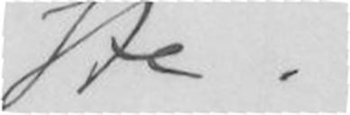ste.
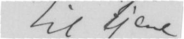til tjene
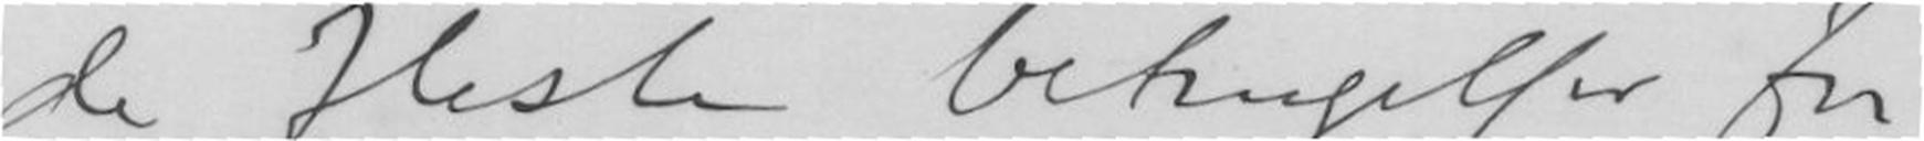de fleste betrufelser for
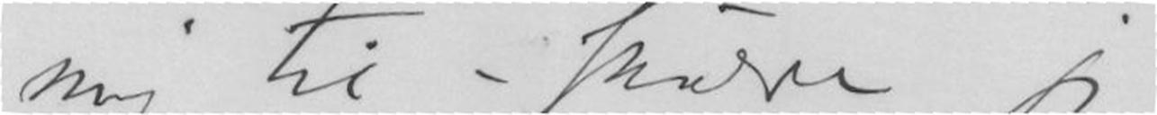mig til - passer jeg
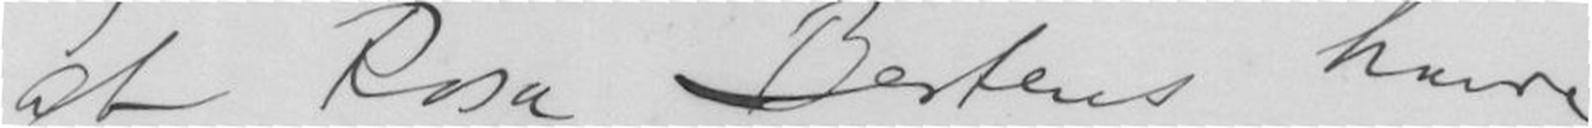at Rosa Bertens havde
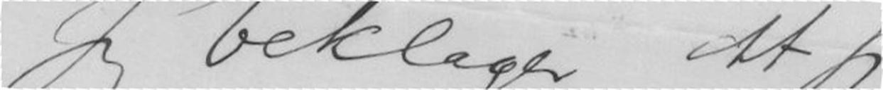Jeg beklager at jeg
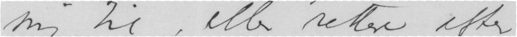mig til, eller rettere efter
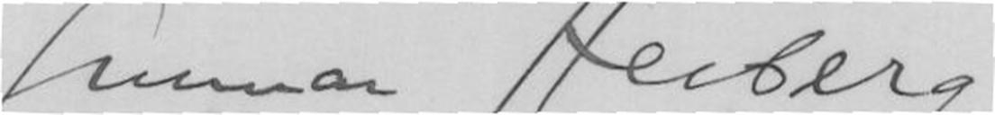Gunnar Heiberg
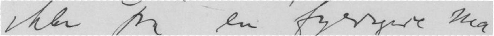ikke på, en fyldigere må-
